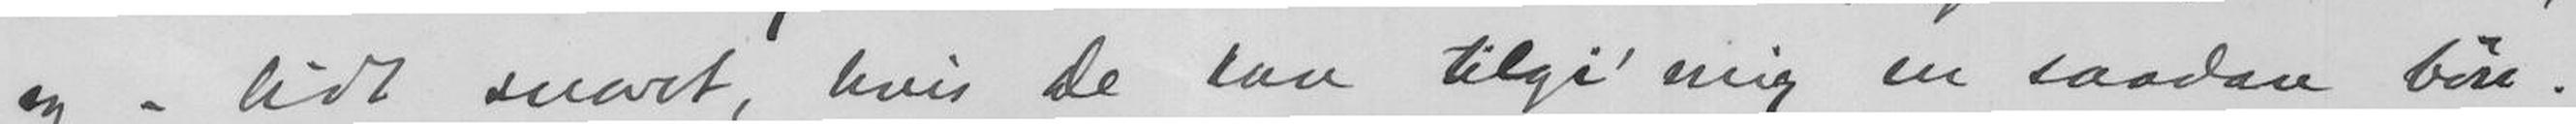og - lidt snart, hvis De kan tilgi mig en saadan bøn.
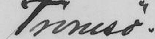TromsøBL.
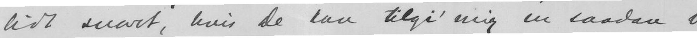min adresse er: Stud. Jur. Bernt Lie, byfogden i
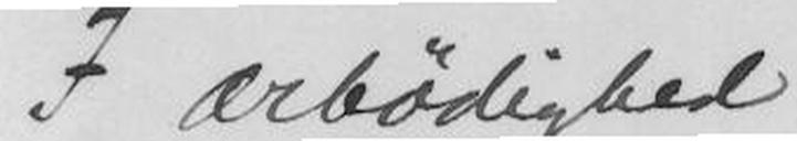I ærbødighed
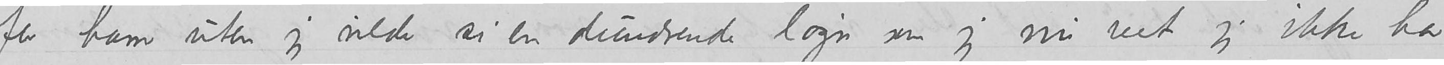for ham uten jeg vilde si en dundrende løgn som jeg nu vet jeg ikke har
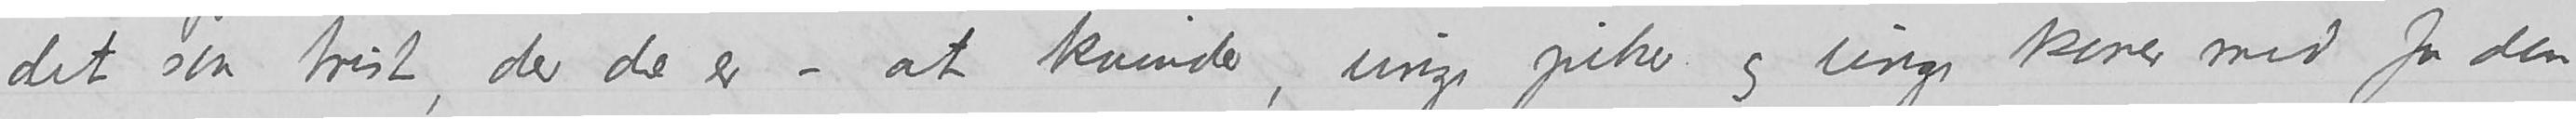det saa trist, der de er – at kvinder, unge piker og unge koner med for den
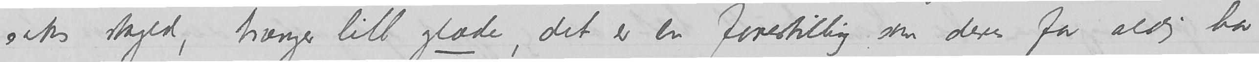saks skyld, trænger litt glæde, det er en forestilling som deres far aldrig har
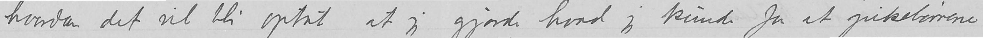hvordan det vil bli optat at jeg gjorde hvad jeg kunde for at pikebørnene
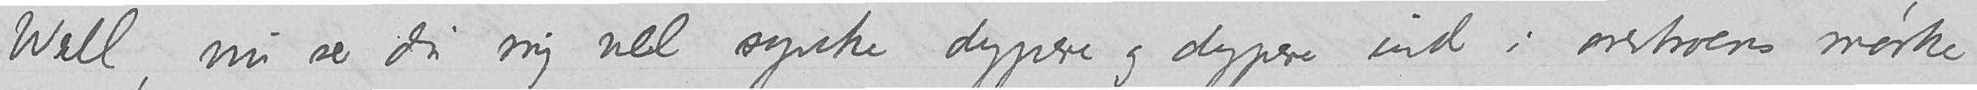Well, nu ser du mig vel synke dypere og dypere ind i overtroens mørke
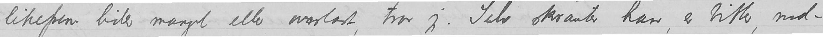likefrem lider mangel eller overlast, tror jeg.  Selv skranter han, er bitter, ned-
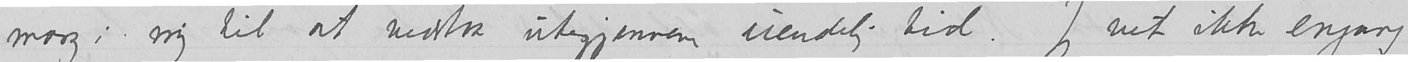marg i mig til at nedstaa utigjennom uendelig tid.  Jeg vet ikke engang
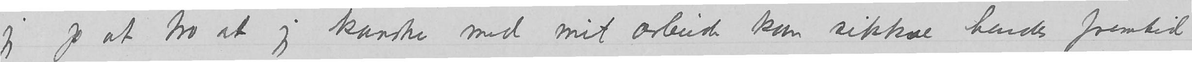jeg jo at tro at jeg kanske med mit arbeide kan sikkre hendes fremtid
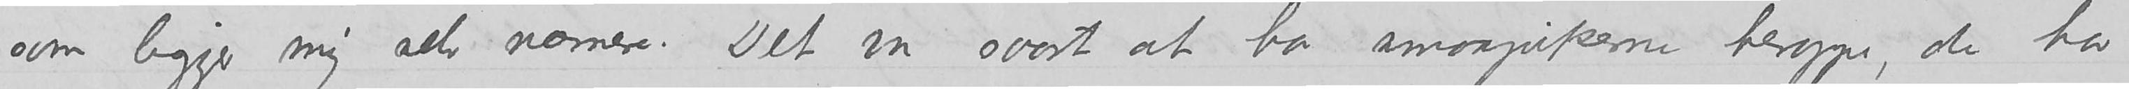som ligger mig selv nærmere.  Det var saart at ha smaapikerne heroppe, de har
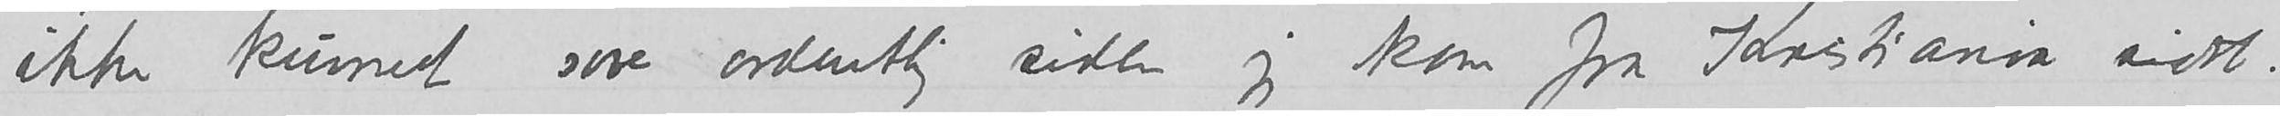ikke kunnet sove ordentlig siden jeg kom fra Kristiania sisst.
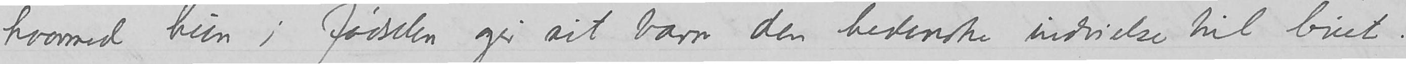, hvormed hun i fødselen gir sit barn den hedenske indvielse til livet.
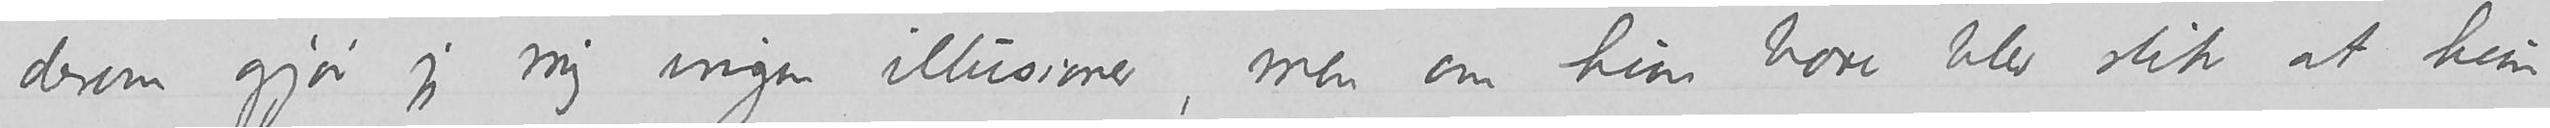derom gjør jeg mig ingen illusioner, men om hun bare blev slik at hun
